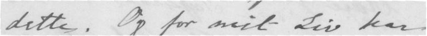dette. Og for mit Liv har
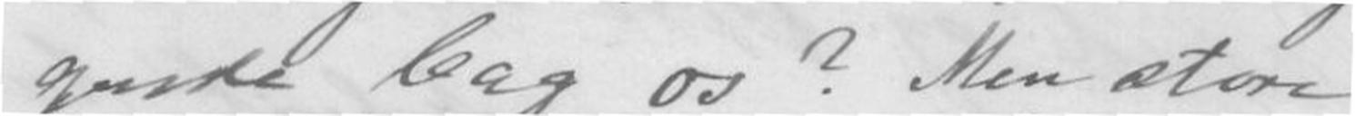gende bag os? Men store
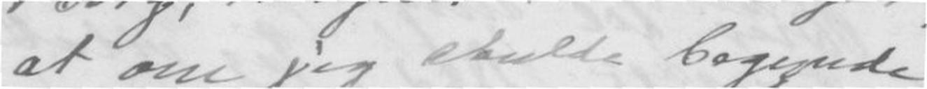at om jeg skulde begynde
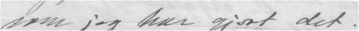som jeg har gjort det.
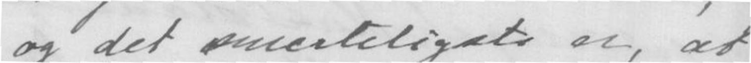og det smerkeligste er, at
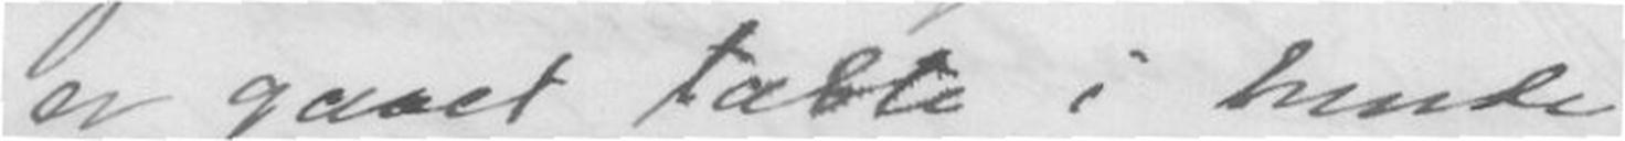er gaaet tabte i hende,
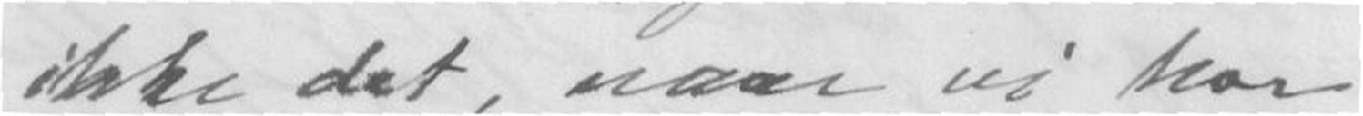ikke det, naar vi har
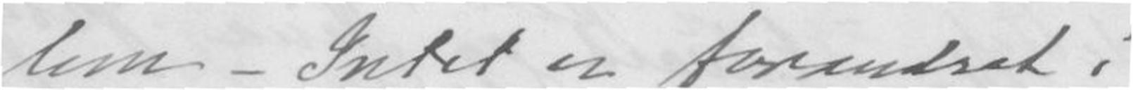lem - Intet er forandret i
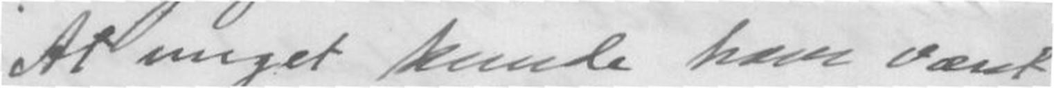At meget kunde have været
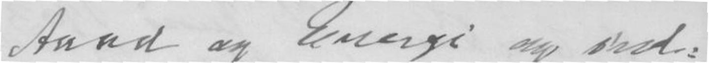Aand og Energi og ind-
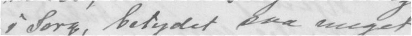i Sorg, betydet saa meget,
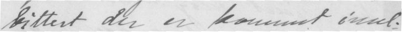bittert der er kommet imel-
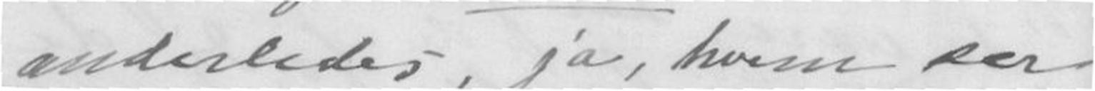anderledes, ja, hvem ser
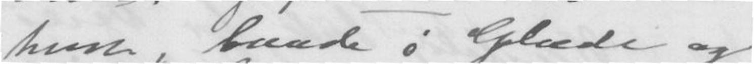hun, baade i Glæde og
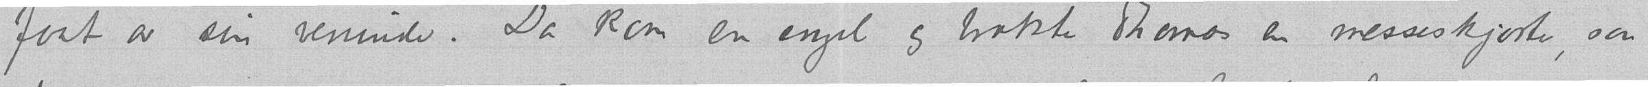faat av sin veninde. Da kom en engel og brakte Thomas en messeskjorte, saa
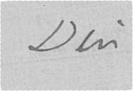Din
